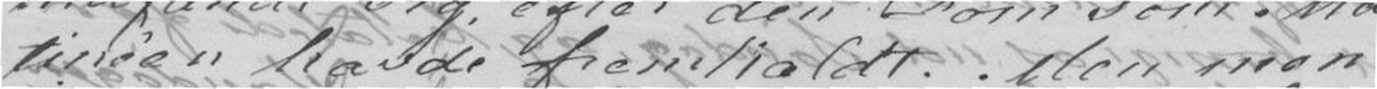tinèen havde fremkaldt. Men mon
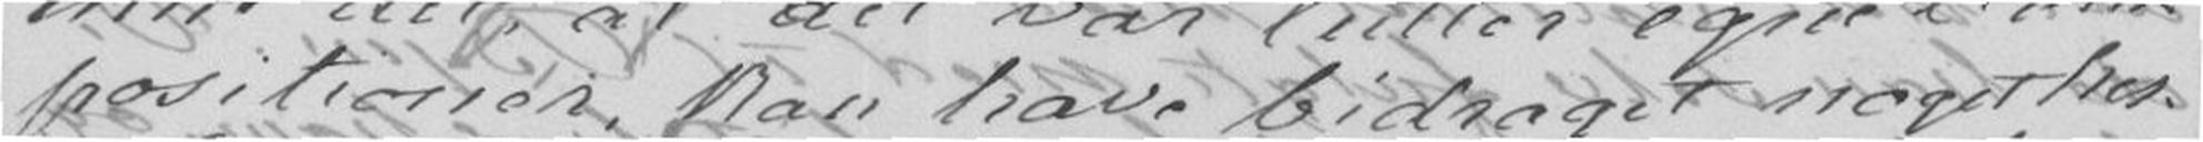positioner, kan have bidraget noget her-
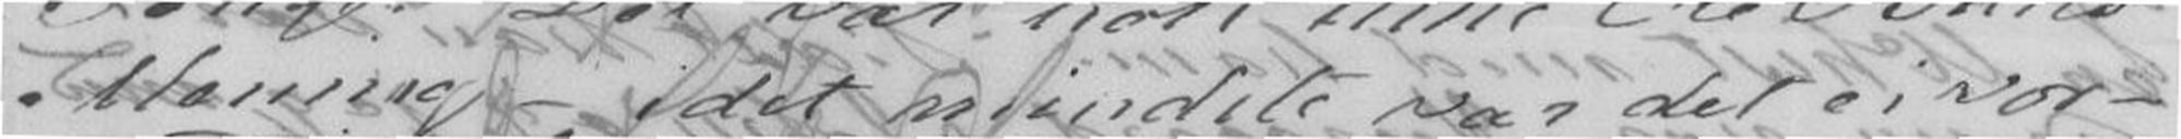Mening i det mindste var det ei vor
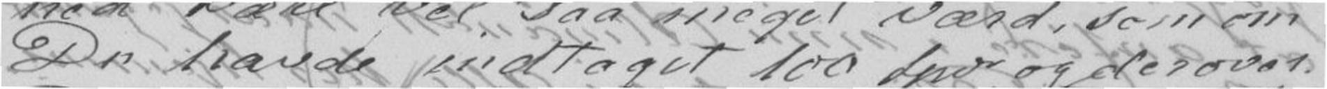Du havde indtaget 100 Spd og derover.
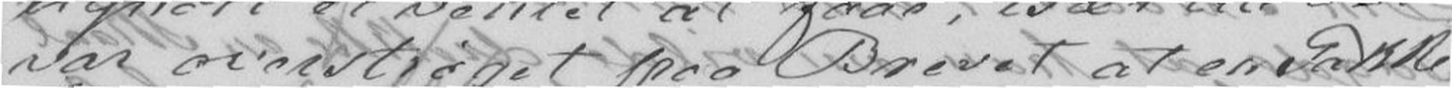var overstrøget paa Brevet at en Pakke
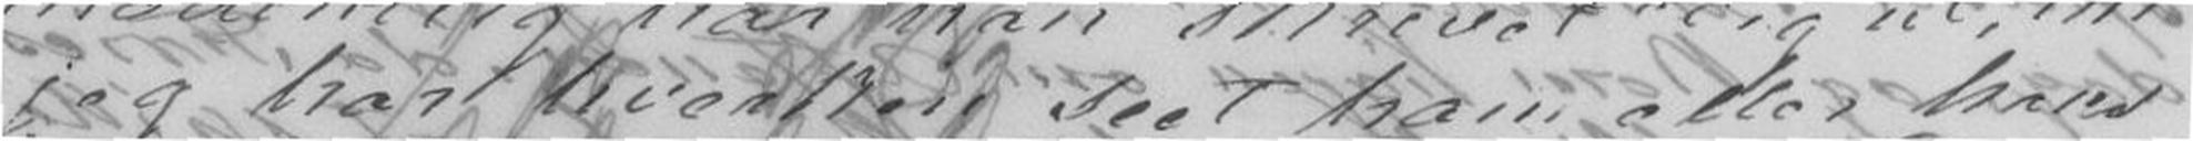jeg har hverken seet ham eller hans
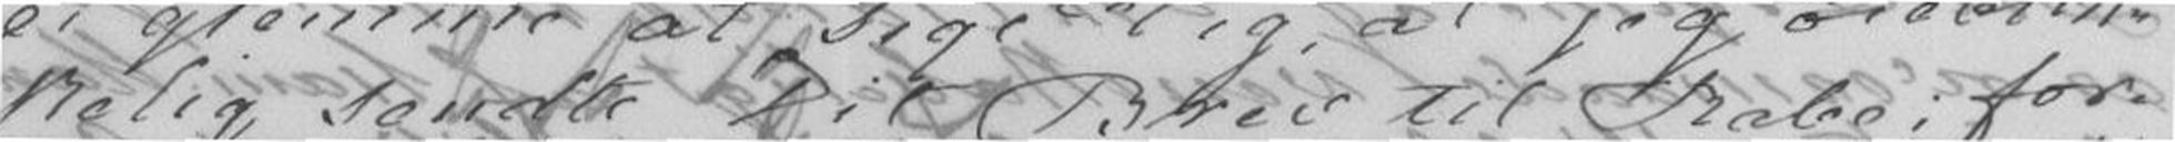kelig sendte Dit Brev til Rabe; for-
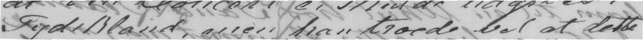Tydskland, men han troede vel at dette
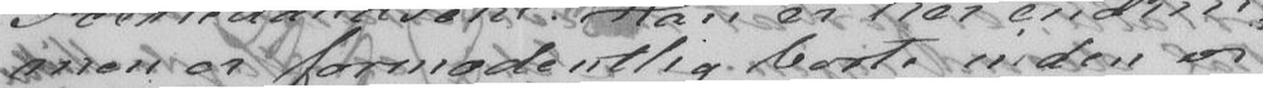men er formodentlig borte inden vi
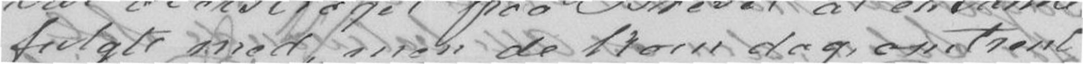fulgte med, men det kom dog omtrent
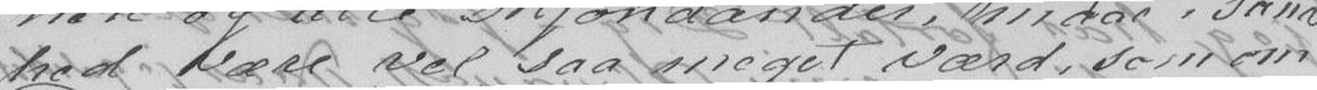hed være vel saa meget værd, som om
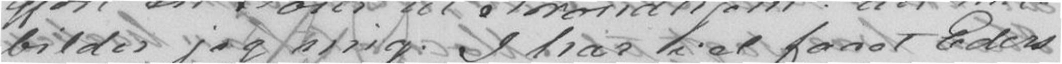bilder jeg mig. I har vel faaet Eders
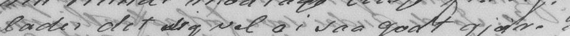lades det sig vel ei saa godt gjøre
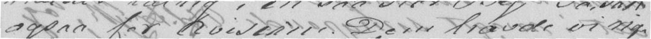ogsaa for hviserne Dem havde vi rig-
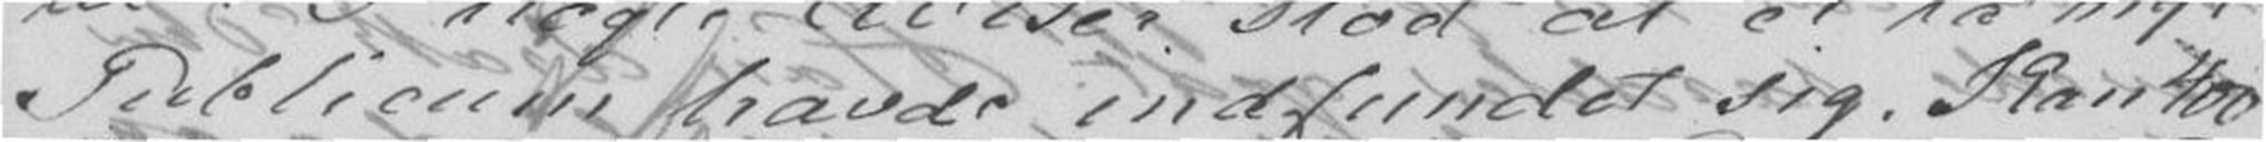Publicum havde indfundet sig. Kan 400
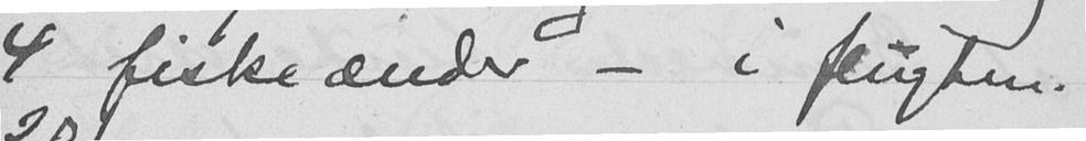4 fiskeænder - i flugten.
